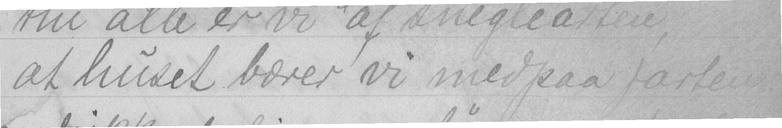at huset bærer vi med paa farten".
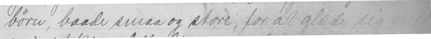børn, baade smaa og store, for at glæde sig med
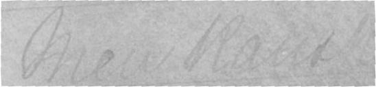Men kanske er
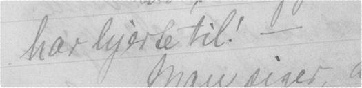har hjerte til! -
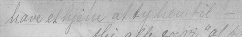have et hjem at ty hen til -
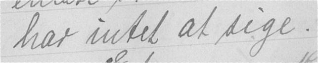har intet at sige.
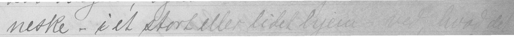neske - i et stort eller lidet hjem ved, hvad det
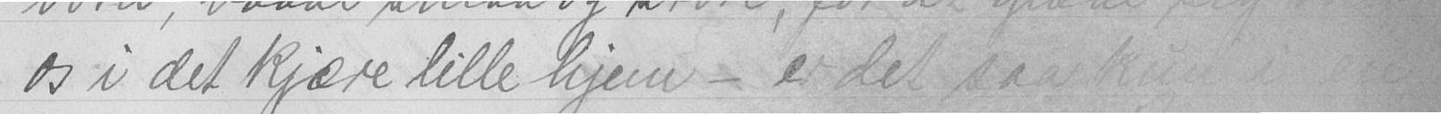os i det kjære lille hjem - er det saa kun i en
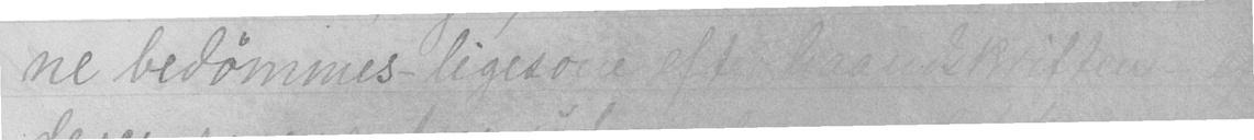ne bedømmes ligesom efter haandskriften og
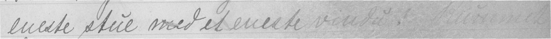eneste stue med et eneste vindu! Rummet
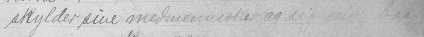skylder sine medmennesker og sig selv, baade
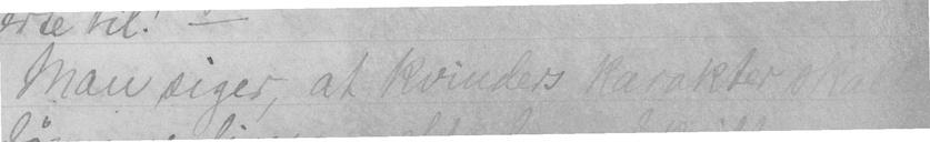Man siger, at kvinders karakter skal -
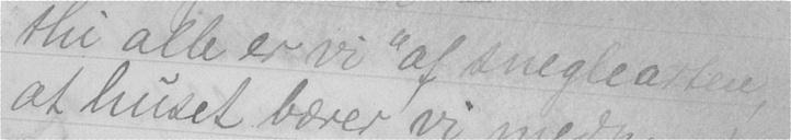thi alle er vi "af sneglearten,
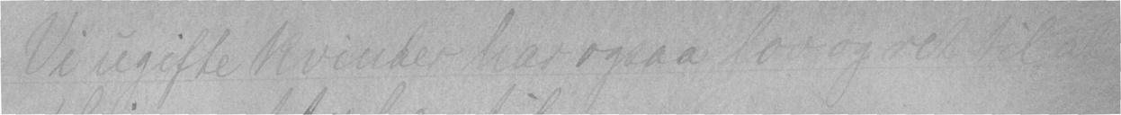Vi ugifte kvinder har ogsaa lov og ret til at
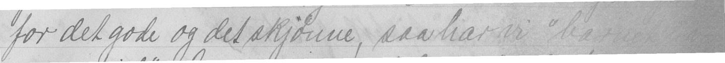for det gode og det skjønne, saa har vi "barne
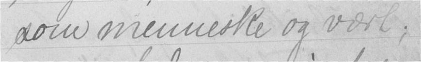som menneske og vært;
